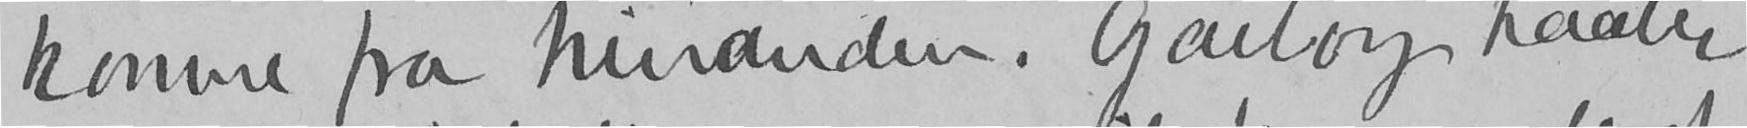komme fra hinanden. Garborg haaber
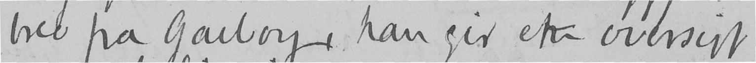brev fra Garborg, han gir en oversigt
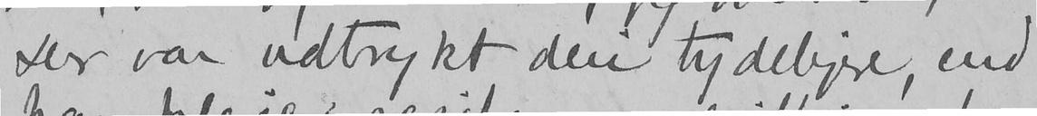Der var udtrykt deri tydeligere, end
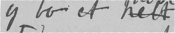og bo et helt
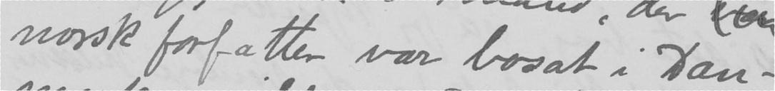norsk forfatter var bosat i Dan-
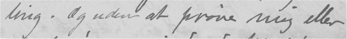ling. Og uden at prøve mig eller
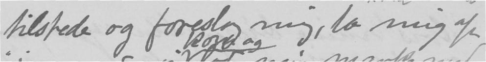tilstede og foreslog mig, lo mig op
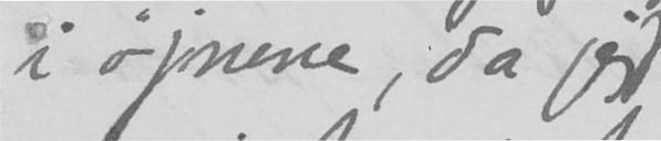i øjnene, da jeg
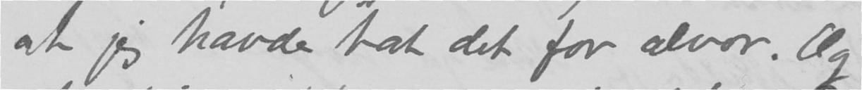at jeg havde tat det for alvor. Og
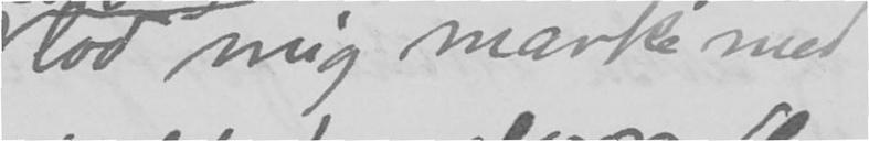lod mig mærke med
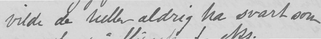vilde de heller aldrig ha svart som
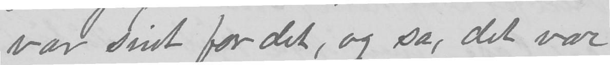var sindt for det, og sa, det var
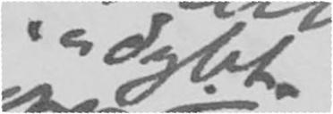"dybt"
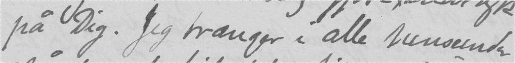på Dig. Jeg trænger i alle henseender
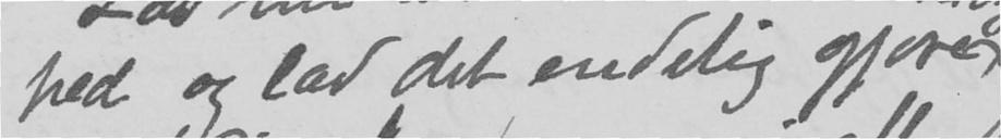hed og lad det endelig gjøre
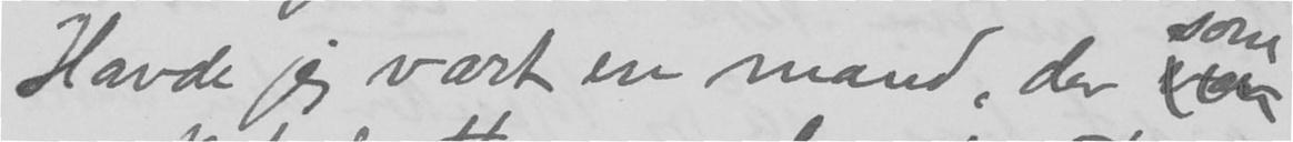Havde jeg vært en mand, der var
